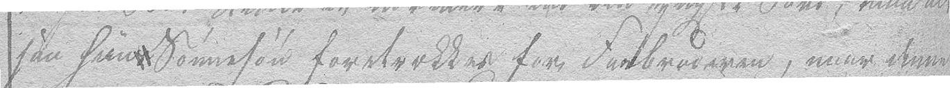saa hans Sønnesøn foretrækkes for Farbroderen, naar denne
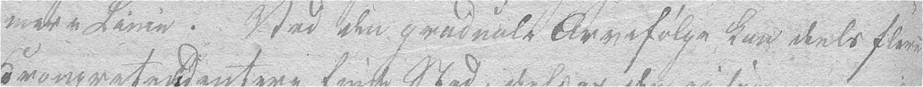mere Linie. Ved den graduale Arvefølge kan deels flere
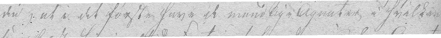den, at i det første have de mandlige Agnater i hvilken
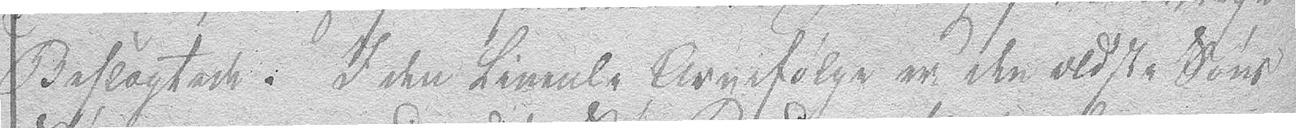Beslægtede. I den Lineale Arvefølge er den ældste Søns
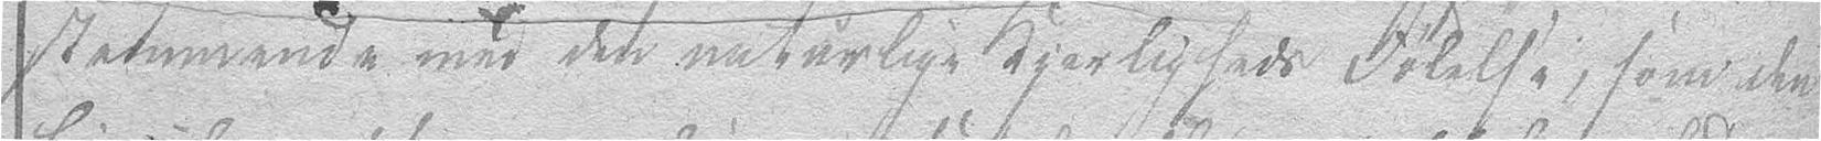stemmende med den naturlige Kjærligheds Følelse, som den
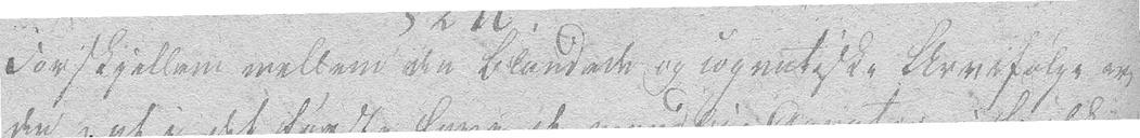Forskjellen mellem den blandede og cognatiske Arvefølge er
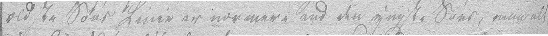ældste Søns Linie er nærmere end den yngste Søns, maa alt-
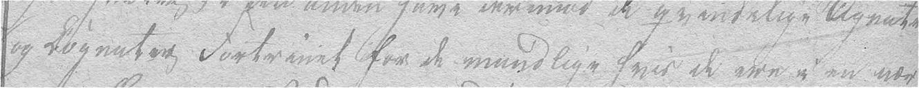og Cognater Fortrinet for de mandlige hvis de ere i en nær-
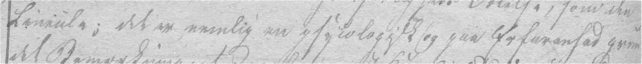Liniale; det er nemlig en psychologisk og paa Erfarenhed grun-
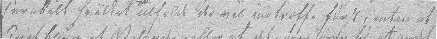surabelt hvilket Tilfælde der vil indtræffe først, enten at
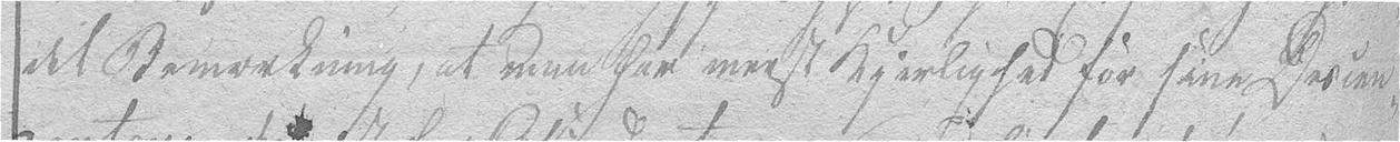det Bemærkning, at man har meest Kjærlighed for sine Descen-
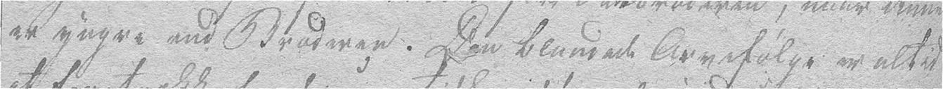er yngre end Broderen. Den blandede Arvefølge er altid
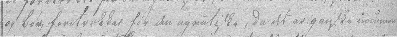og bør foretrækkes før den agnatiske, da det er ganske incommen-
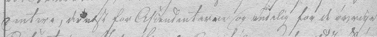dentere, dernæst for Ascendenterne og endelig for de øvrige
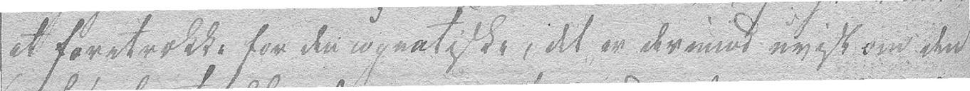at foretrække for den cognatiske, det er derimod uvist om den
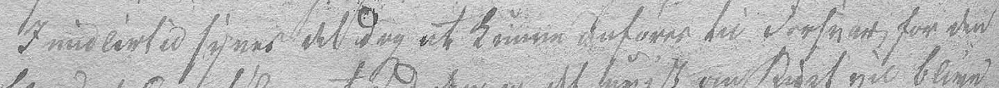Imidlertid synes det dog at kunne anføres til Forsvar for den
